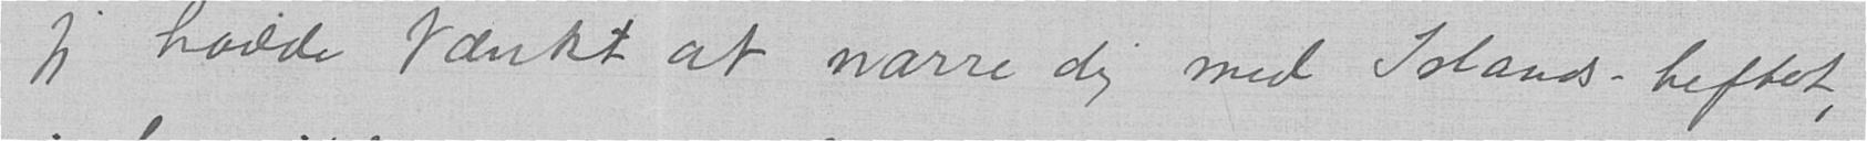jeg hadde tænkt at narre dig med Islands-heftet,
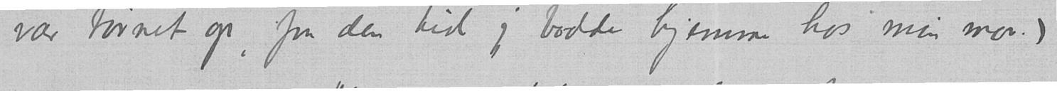var tørnet op, fra den tid jeg bodde hjemme hos min mor.)
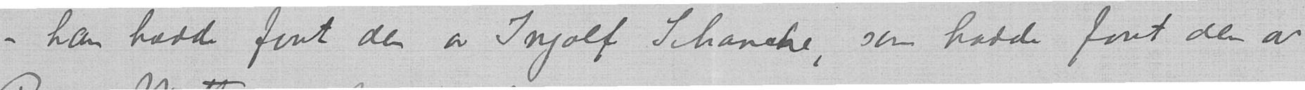- han hadde faat den av Ingolf Schanche, som hadde faat den av
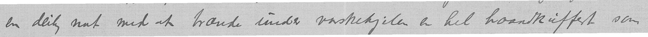en deilig nat med at brænde under vaskekjelen en hel haandkuffert som
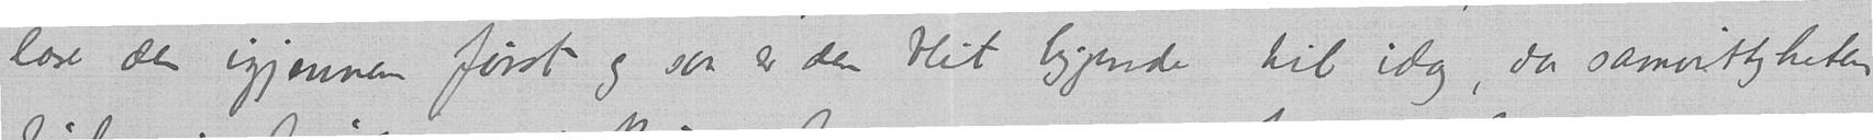læse den igjennem først og saa er den blit liggende til idag, da samvittigheten
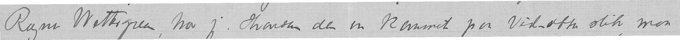Ragna Wettergreen, tror jeg. Hvordan den var kommet paa vid-otta slik, maa
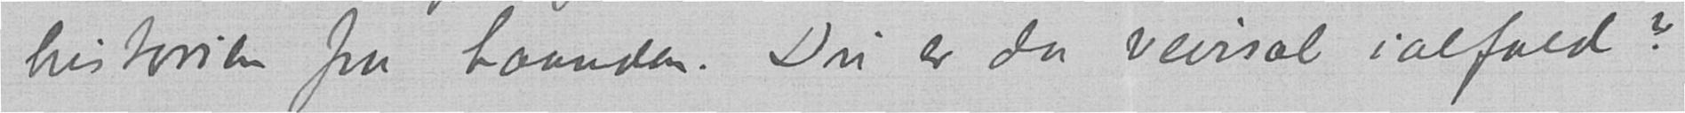historien fra haanden. Du er da veirsæl ialfald?
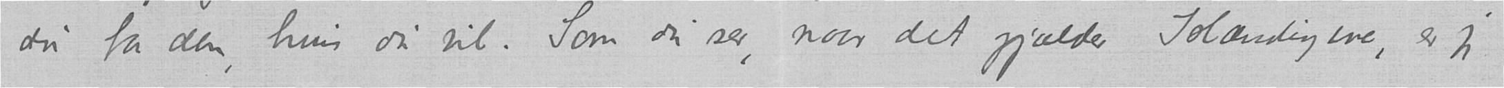du ta den, hvis du vil. Som du ser, naar det gjælder Islændingene, er jeg
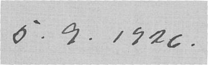5.9.1926.
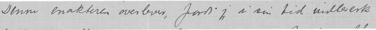Denne enakteren overlever, fordi jeg i sin tid indleverte
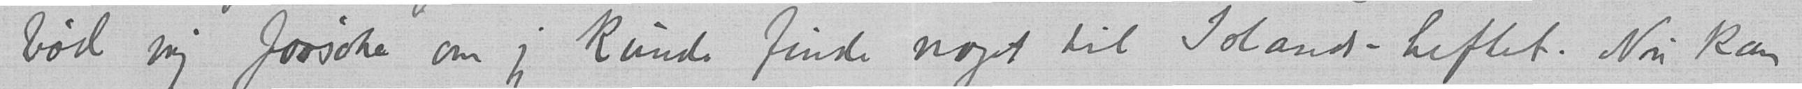bød mig forsøke om jeg kunde finde noget til Islands-heftet. Nu kan
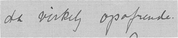da virkelig opofrende.
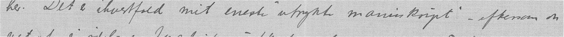her. Det er ihvertfald mit eneste "utrykte manuskript" – eftersom du
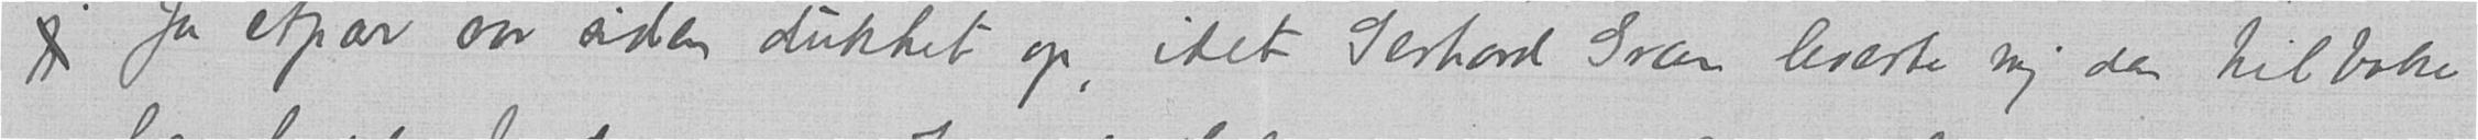jeg for etpar aar siden dukket op, idet Gerhard Gran leverte mig den tilbake
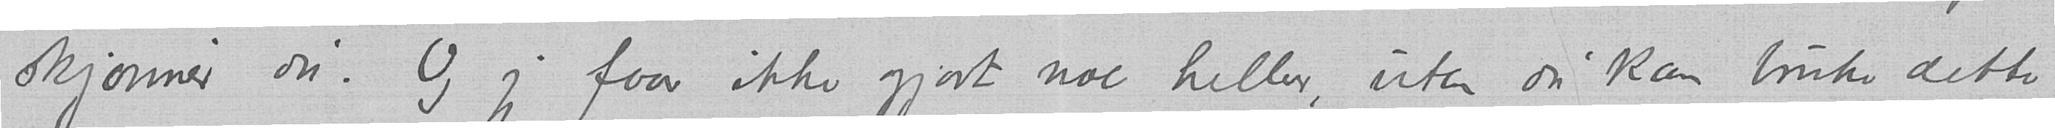skjønner du. Og jeg faar ikke gjort noe heller, uten du kan bruke dette
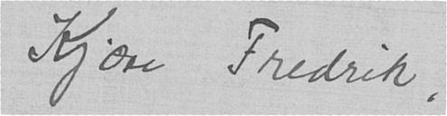Kjære Fredrik,
